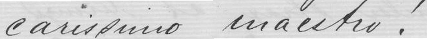carissemo maestro!
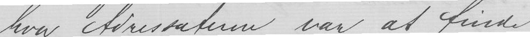hva Adressaterne var at finde,
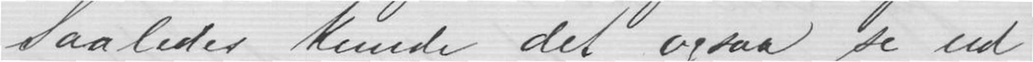Saaledes kunde det ogsaa se ud
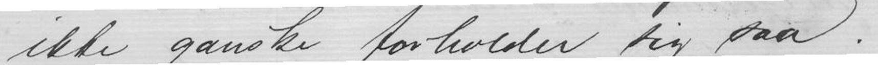ikke ganske forholder sig saa.
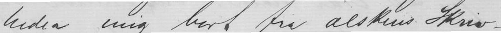heder mig bort fra alskens Skriv-
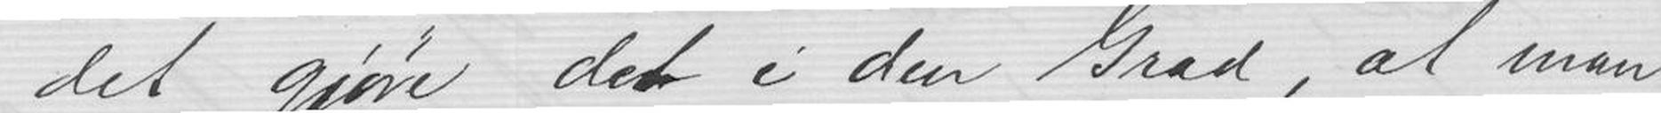det gjøre det i den Grad, at man
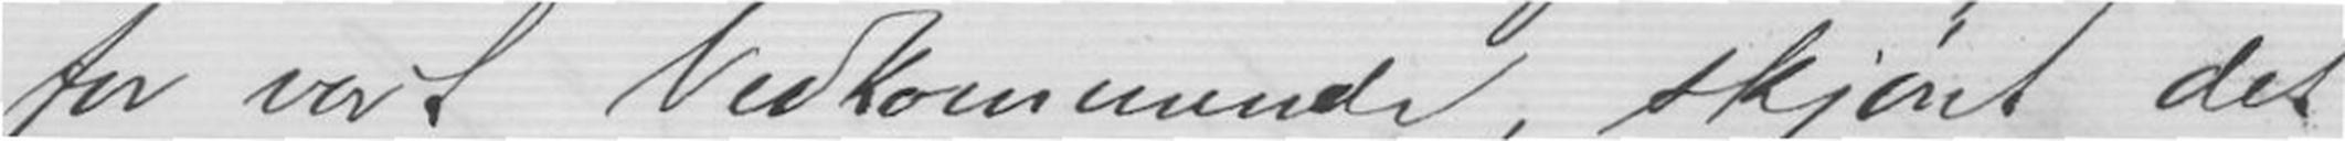for vort Vedkommende, skjønt det
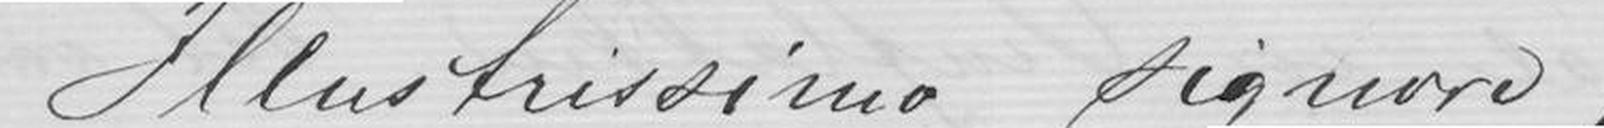Illustrissimo signore,
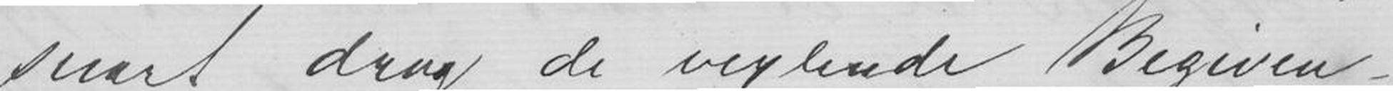snart drog de vexlende Begiven-
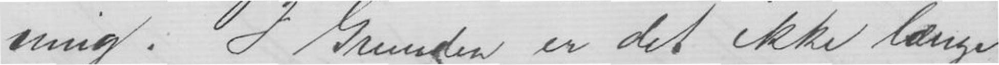ning. I Grunden er det ikke længe
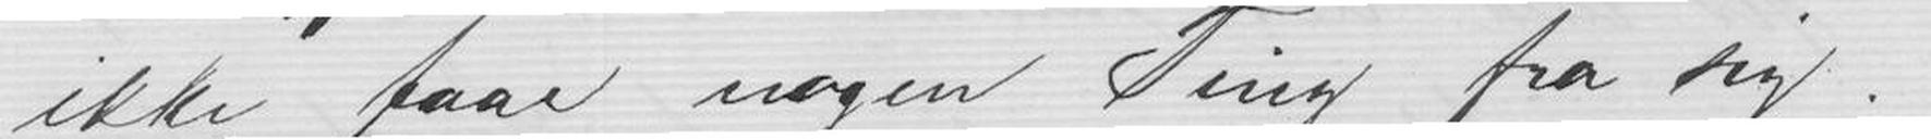ikke faar nogen Ting fra sig.
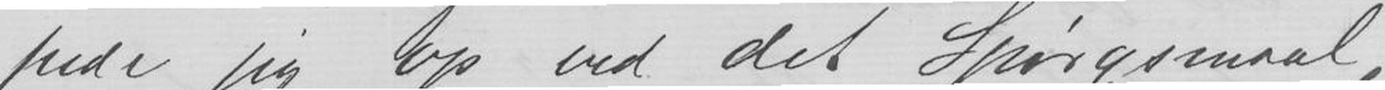pede jeg op ved det Spørgsmaal,
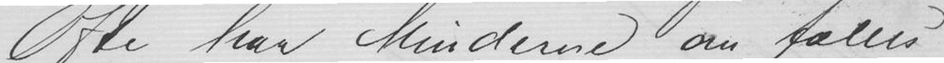Ofte har Minderne om fælles
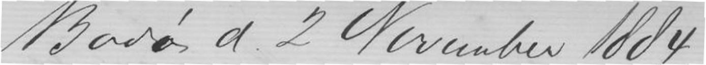Bodø d 2 November 1884.
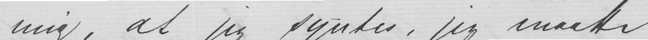mig, at jeg syntes, jeg maatte
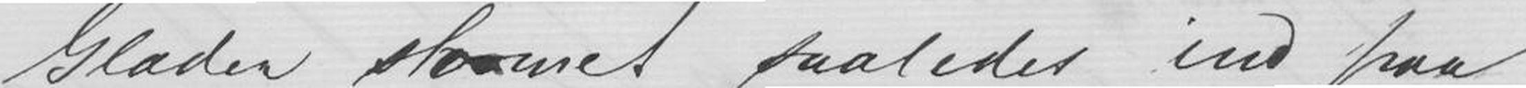Glæder stormet saaledes ind paa
